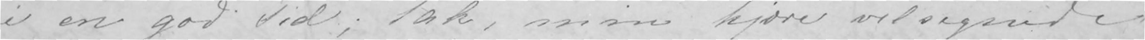i en god Tid; Tak, min kjære velsignede
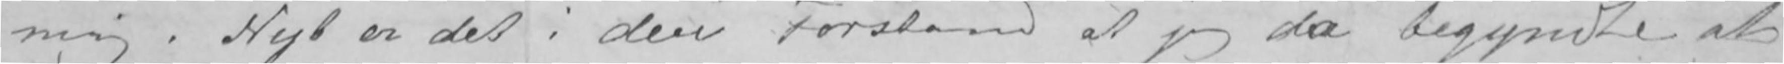mig. Nyt er det i den Forstand at jeg da begyndte at
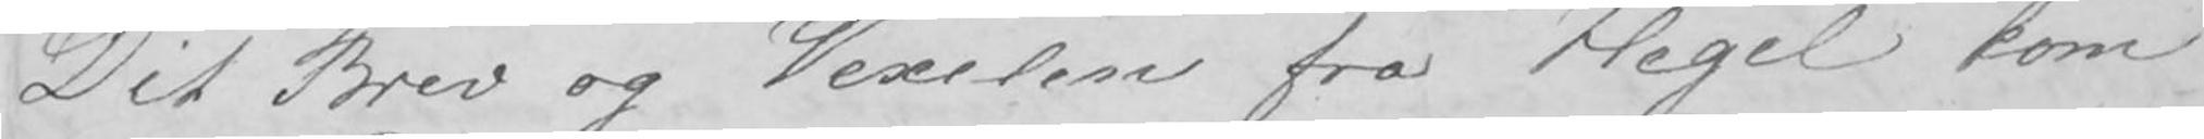Dit Brev og Vexelen fra Hegel kom
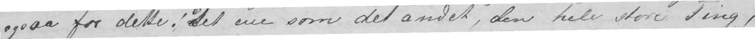ogsaa for dette! Detdet ene som det andet, den hele store Ting,
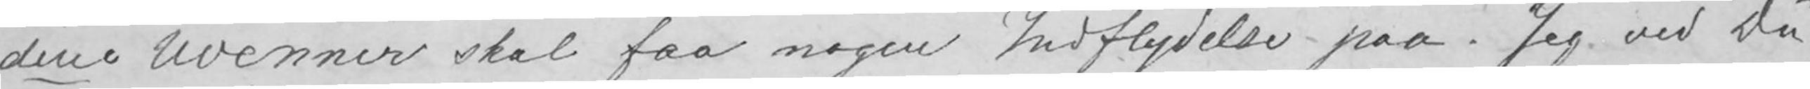dine Uvenner skal faa nogen Indflydelse paa. Jeg ved Du
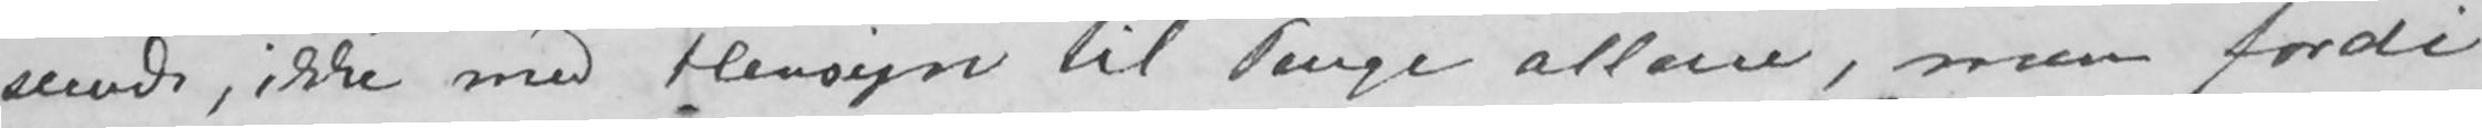seende, ikke med Hensyn til Penge allene, men fordi
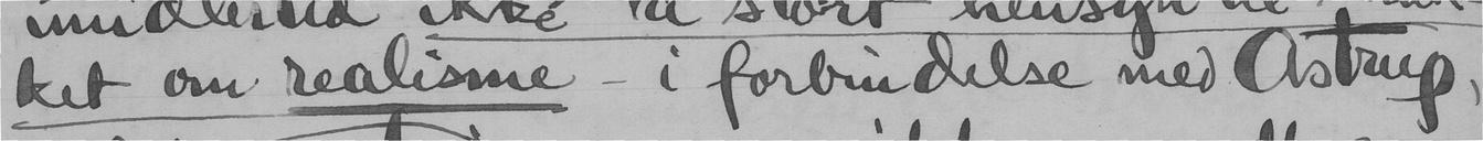ket om realisme - i forbindelse med Astrup,
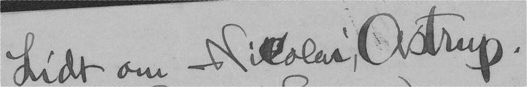Lidt om Nicolai Astrup.
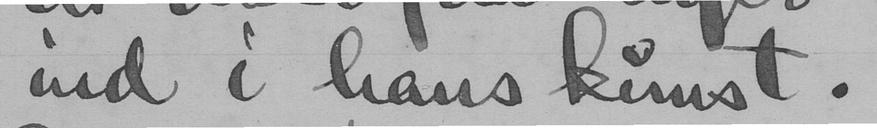ind i hans kunst.
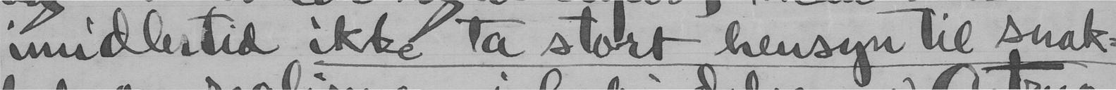imidlertid ikke ta stort hensyn til snak-
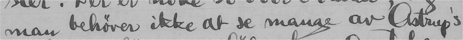man behøver ikke at se mange av Astrup's
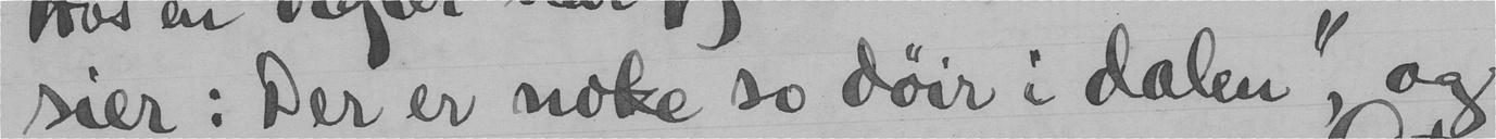sier: Der er noke so døir i dalen", og
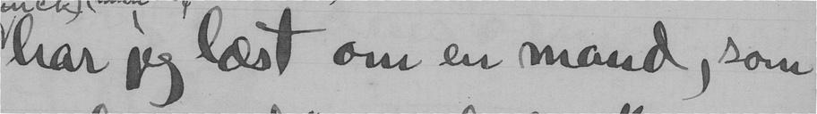har jeg læst om en mand, som
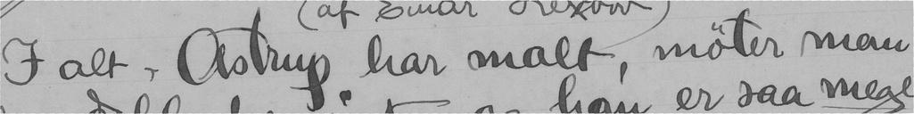I alt, Astrup har malt, møter man
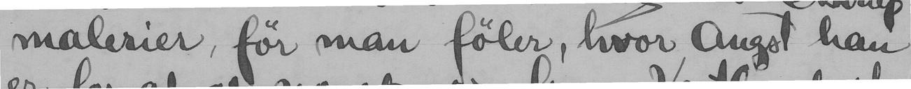malerier, før man føler, hvor angst han
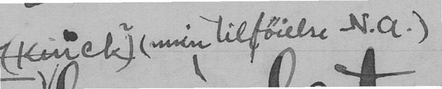(Kinck) (min tilføielse N.A.)
